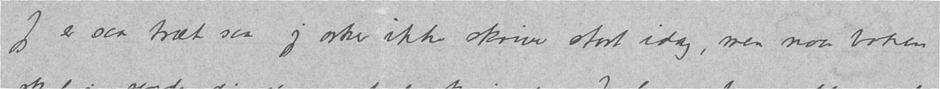Jeg er saa træt saa jeg orker ikke skrive stort idag, men naar boken
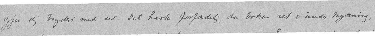gjør dig Bryderi med det. Det haster forfærdelig, da boken alt er under trykning,
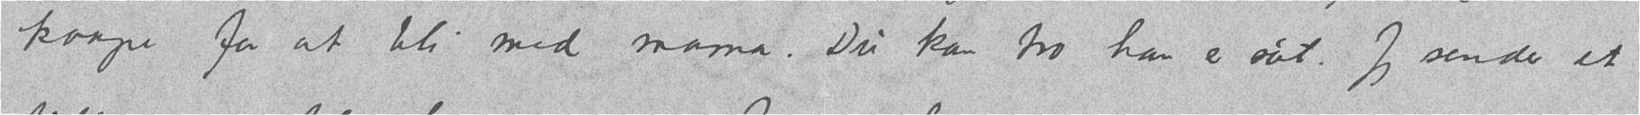kaape for at bli med mama. Du kan tro han er søt. Jeg sender et
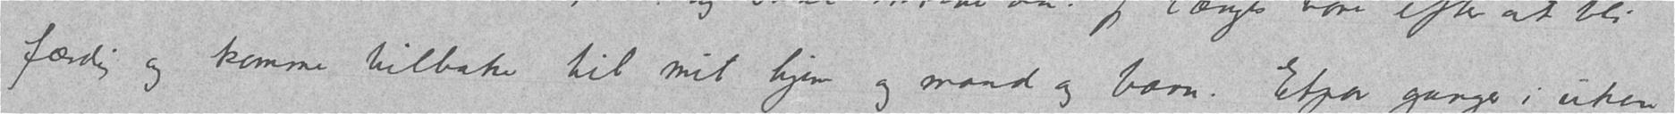færdig og komme tilbake til mit hjem og mand og barn. Etpar gange i uken
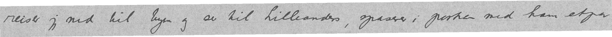reiser jeg ned til byen og ser til Lilleanders, spaserer i parken med ham etpar
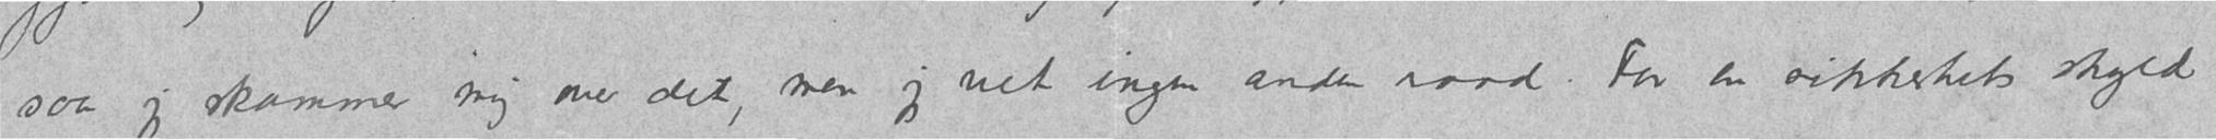saa jeg skammer mig over det, men jeg vet ingen anden raad. For en sikkerhets skyld
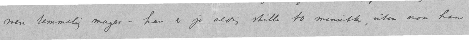men temmelig mager - han er jo aldrig stille to minutter, uten naar han
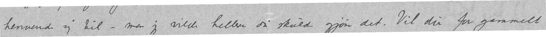henvende sig til - men jeg vilde heller du skulde gjøre det. Vil du for gammelt
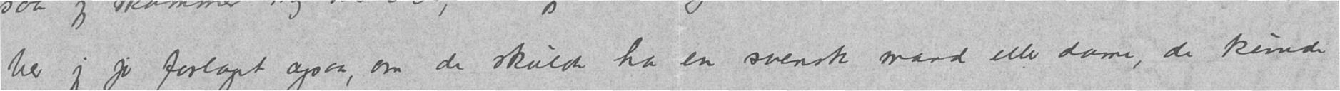har jeg jo forlaget ogsaa, om de skulde ha en svensk mand eller dame, de kunde
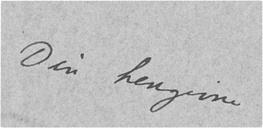Din hengivne
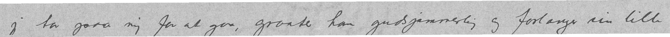jeg tar paa mig for at gaa, graate han gudsjammerlig og forlanger sin lille
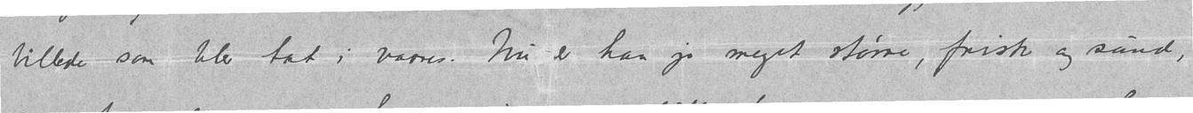billede som ble tat i vaares. Nu er han jo meget større, frisk og sund,
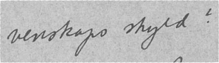venskaps skyld?
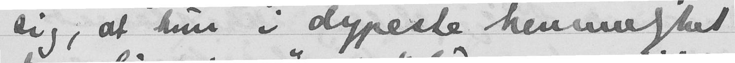sig, at hun i dypeste hemmelighet
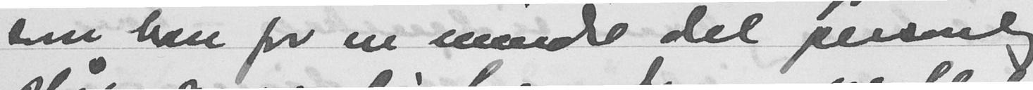som han for en mindre del personlig
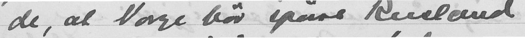de, at Norge bør sprørre Rusland
